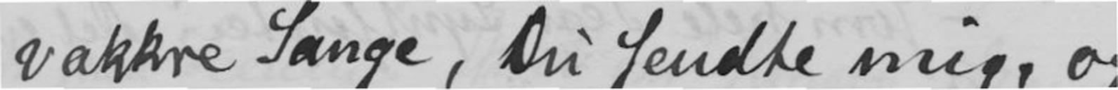vakkre Sange, Du sendte mig, og
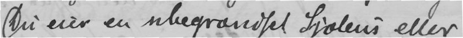Du eier en ubegrændset Sjælens eller
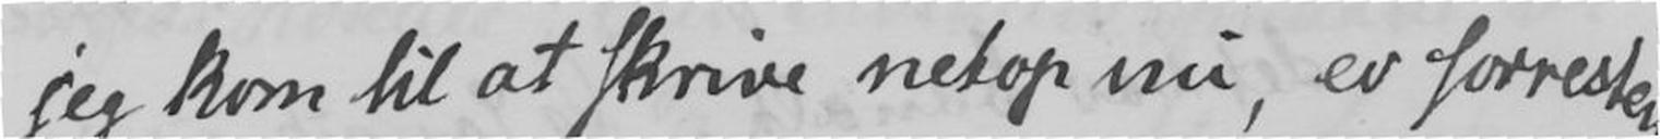jeg kom til at skrive netop nu, er forresten,
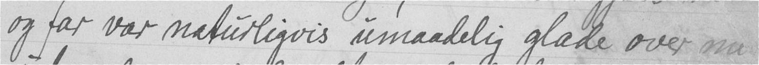og far var naturligvis umaadelig glade over mi
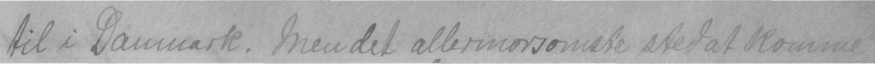til i Danmark. Men det allermorsomste sted at komme
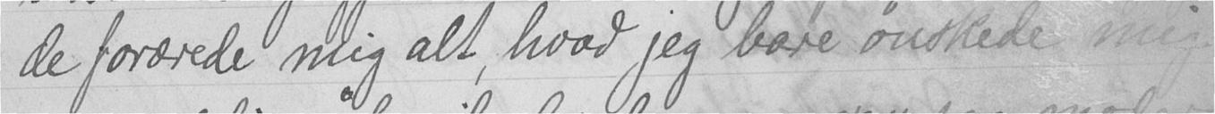de forærede mig alt, hvad jeg bare ønskede mig.
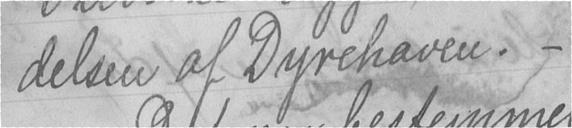delsen af Dyrehaven. -
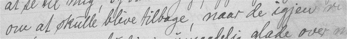om at skulle blive tilbage, naar de igjen re
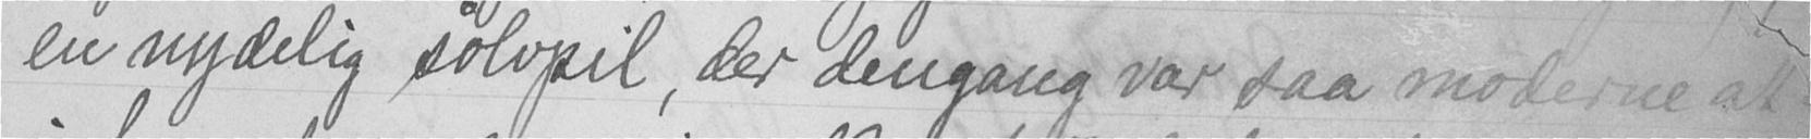en nydelig sølvpil, der dengang var saa moderne at
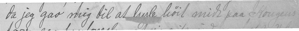da jeg gav mig til at hyle høit midt paa Kongens
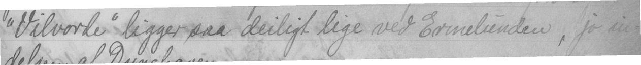"Vilvorde" ligger saa deiligt lige ved Ermelunden,  jo in-
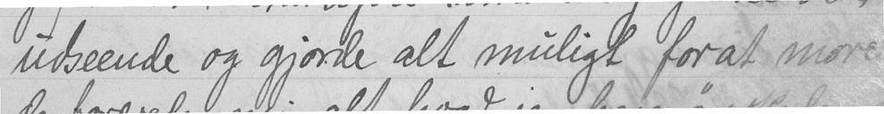udseende og gjorde alt muligt for at more
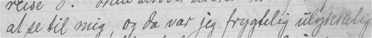at se til mig, og da var jeg frygtelig ulykkelig
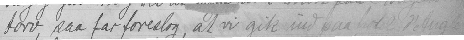torv, saa far foreslog, at vi gik ind paa hotel d'Angle-
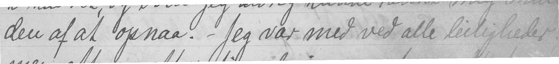den af at opnaa. - Jeg var med ved alle leiligheder
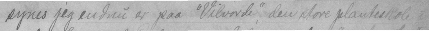synes jeg endnu er paa "Vilvorde", den store planteskole i
